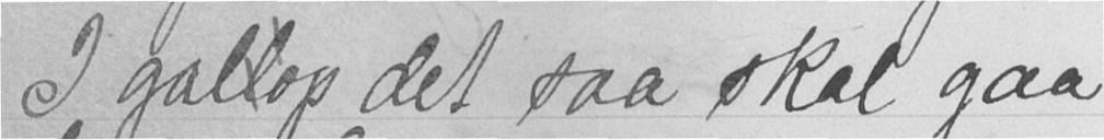I gallop det saa skal gaa
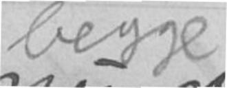begge
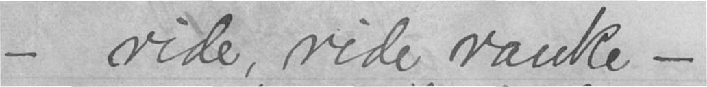- ride, ride ranke -
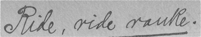Ride, ride ranke.
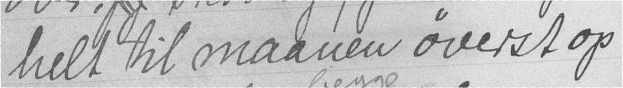helt til maanen øverst op
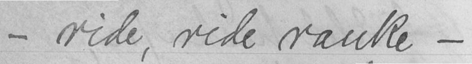- ride, ride ranke -
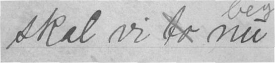skal vi to nu
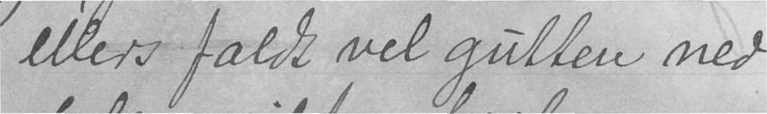ellers faldt vel gutten ned
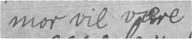mor vil være
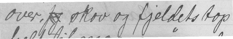over, jog skov og fjeldets top
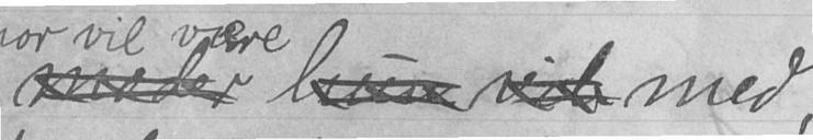moder hun vil med,
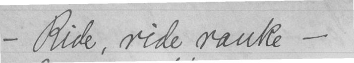- Ride, ride ranke -
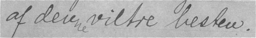af denne viltre hesten.
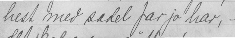hest med sadel far jo har, -
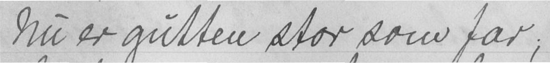nu er gutten stor som far;
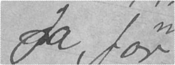Ja, for
